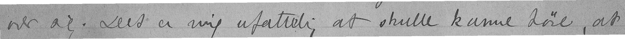over sig. Det er mig ufattelig at skulle kunne døie, at
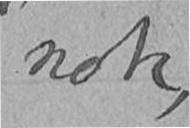nok,
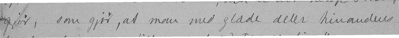gjør, som gjør, at man med glæde deler hinandens
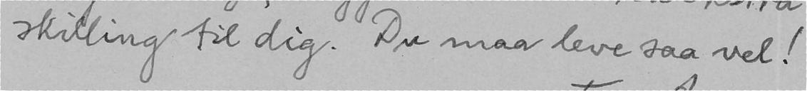skilling til dig. Du maa leve saa vel!
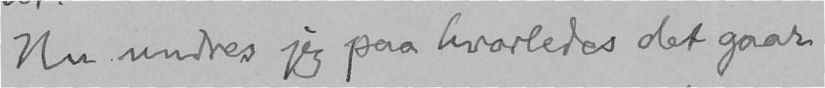Nu undres jeg paa hvorledes det gaar
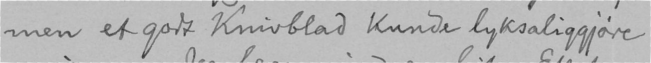men et godt Knivblad kunde lyksaliggjøre
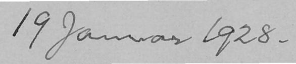19 Januar 1928.
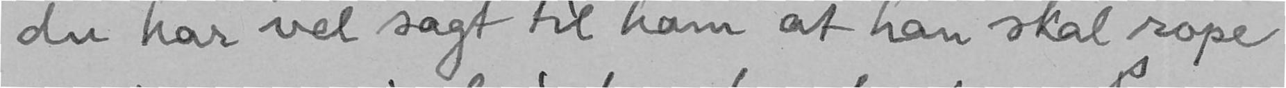du har vel sagt til ham at han skal rope
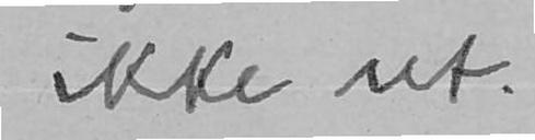ikke ut.
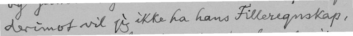derimot vil jeg ikke ha hans Filleregnskap,
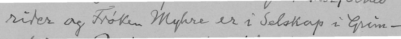rider og Frøken Myhre er i Selskap i Grim-
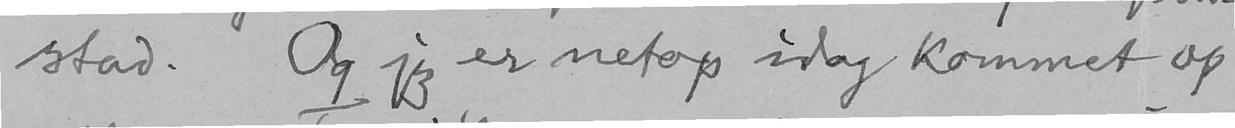stad. Og jeg er netop idag kommet op
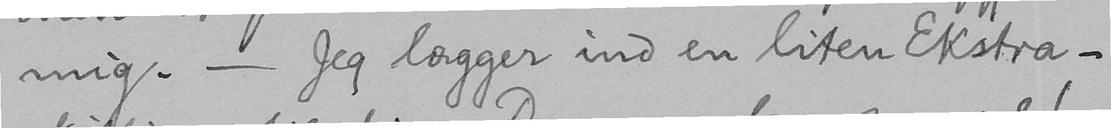mig. - Jeg lægger ind en liten Ekstra-
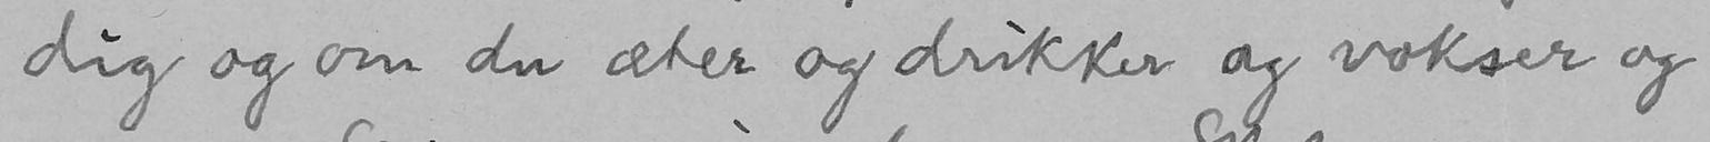dig og om du æter og drikker og vokser og
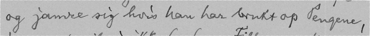og jamre sig hvis han har brukt op Pengene,
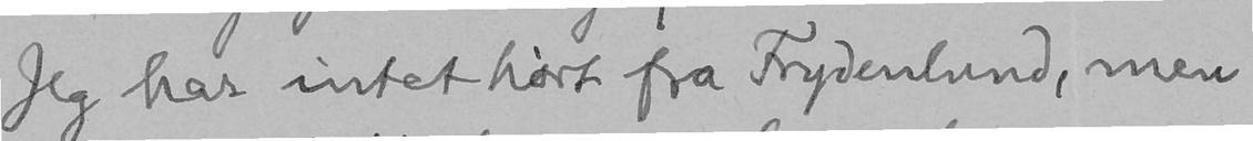Jeg har intet hørt fra Frydenlund, men
